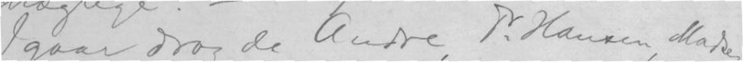Igaar drog de Andre, Dr Hansen, Madsen
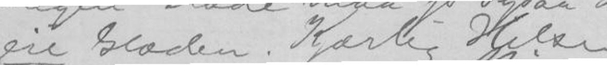eie Glæden. Kjærlig Hilsen
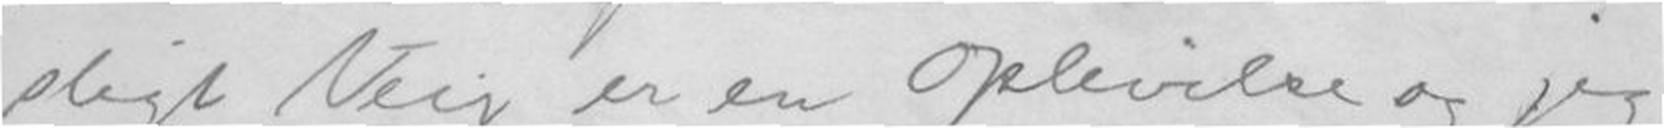sligt Veir er en Oplevelse og jeg
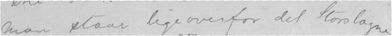man staar ligeover det Storslagne,
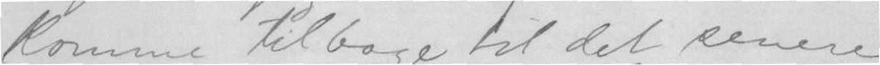komme tilbage til det senere
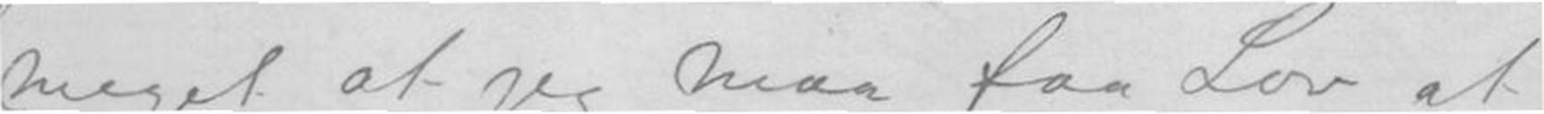meget at jeg maa faa Lov at
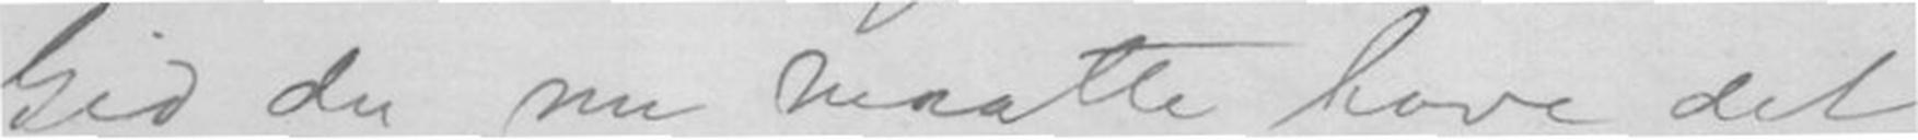Gid du nu maatte have det
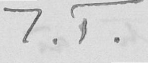J.T.
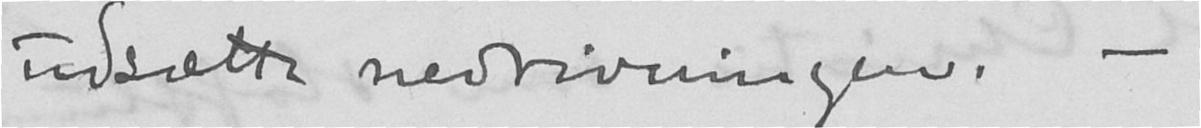udsætte nedrivningen. -
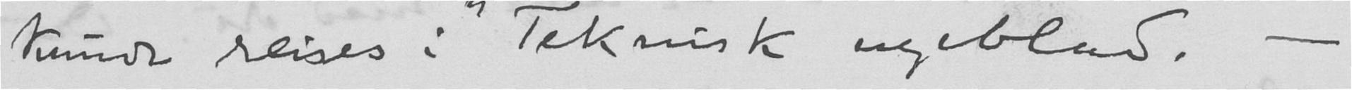kunde reises i "Teknisk ugeblad." -
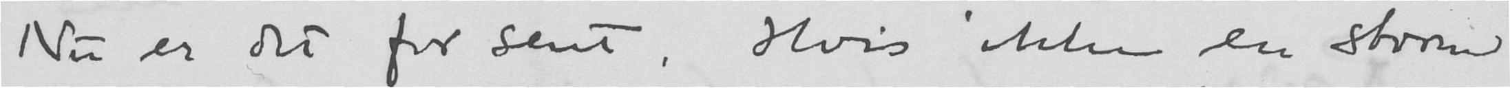Nu er det for sent. Hvis ikke en storm
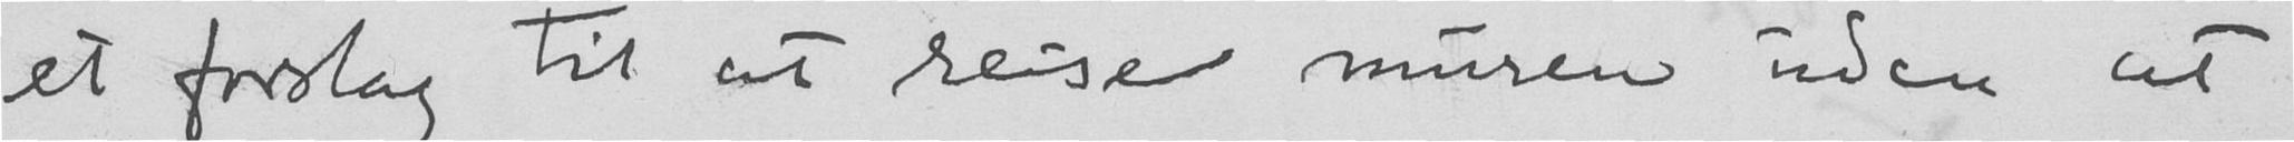et forslag til at reise muren uden at
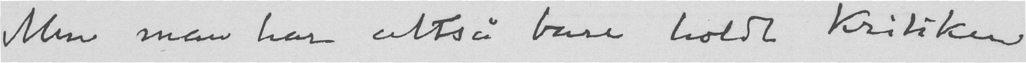Men man har altså bare holdt kritiken
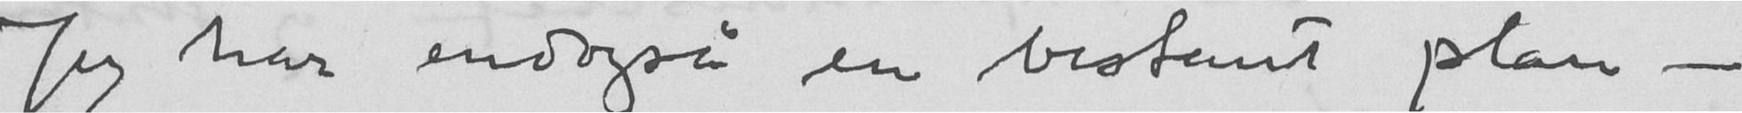Jeg har endogså en bestemt plan -
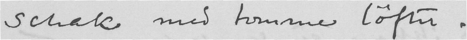schak med tomme løfter.
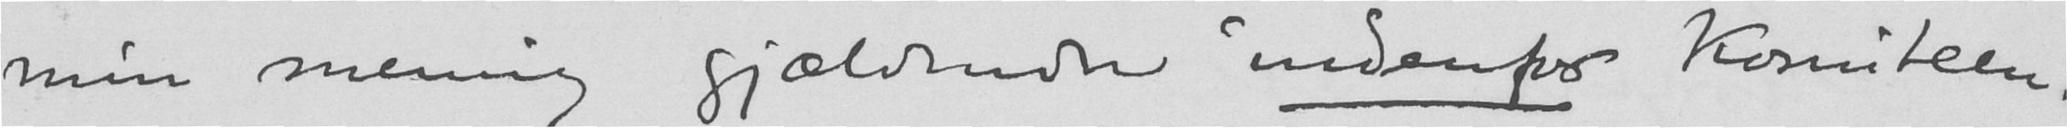min mening gjældende indenfor komiteen.
